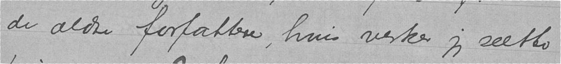de ældre forfattere, hvis verker jeg sætter
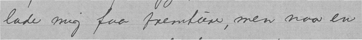lade mig faa fremture, men naar en
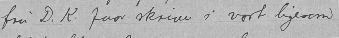fru D.K. faar skrive i vort ligesom
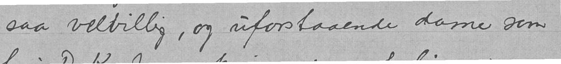saa velvillig, og uforstaaende dame som
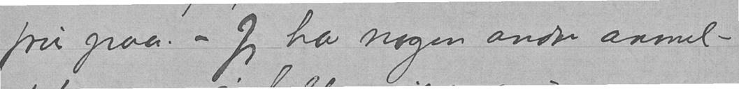pris paa. - Jeg har nogen andre anmeld-
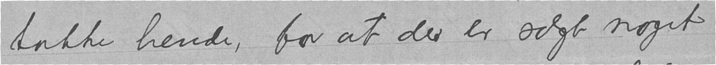takke hende, for at der er solgt noget.
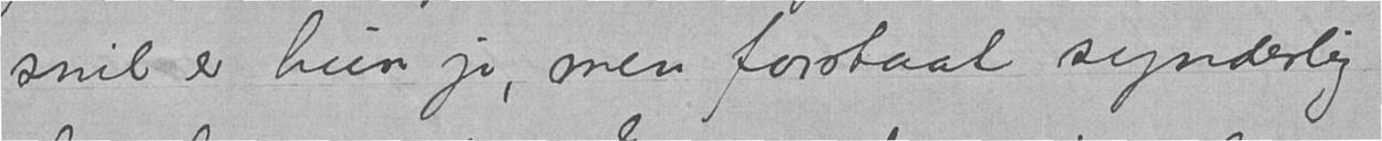snil er hun jo, men forstaat synderlig
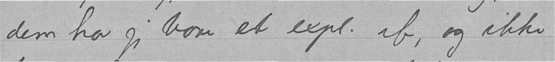dem har jeg bare et expl. af, og ikke
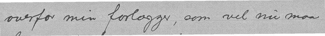overfor min forlægger, som vel nu maa
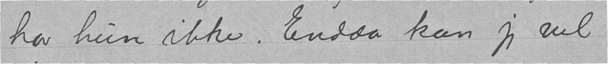har hun ikke. Endda kan jeg vel
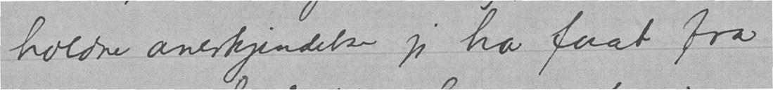holdne anerkjendelse jeg har faat fra
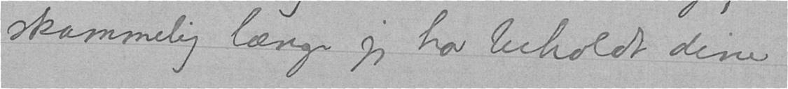skammelig længe jeg har beholdt dine
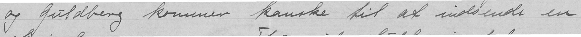og Guldberg kommer kanske til at indsende en
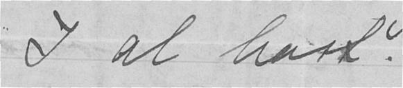I al hast.
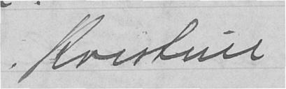Kristine
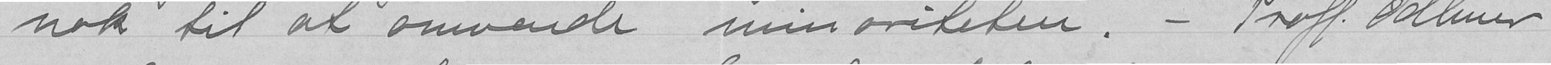nok til at omvende minoriteten. - Proff. Odhner
